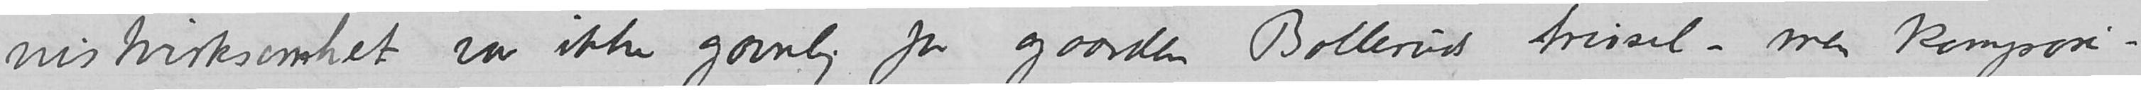istvirksomhet var ikke gavnlig for gaarden Bolleruds trivsel – men komposi
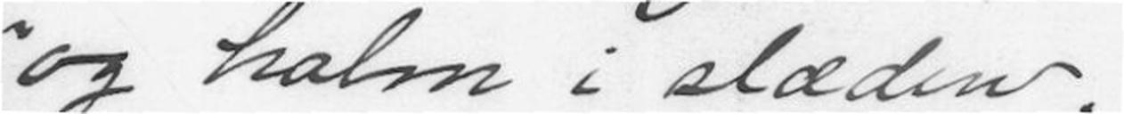"og halm i slæden."
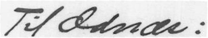Til Odnæs:
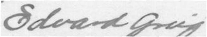Edvard Grieg.
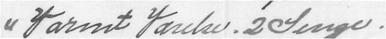"Varmt Værelse. 2 Senge.
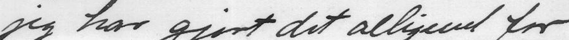jeg har gjort det alligevel for
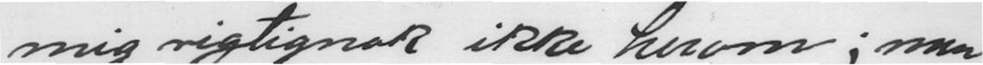mig rigtignok ikke herom; men
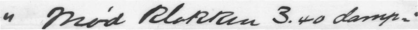" Mød klokken 3.40 damp-"
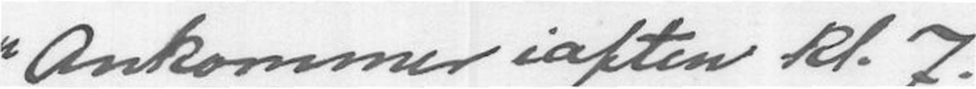"Ankommer iaften kl 7.
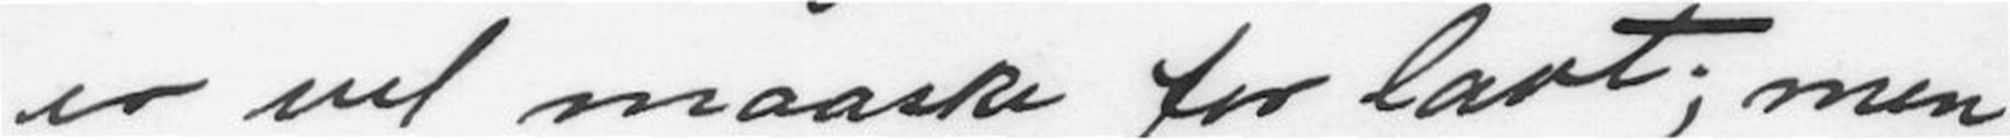er vel maaske for lavt; men
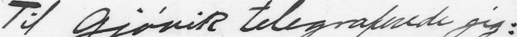Til Gjøvig telegraferede jeg:
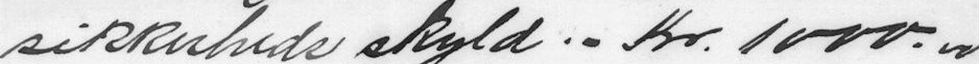sikkerheds skyld. - Kr. 1000.00
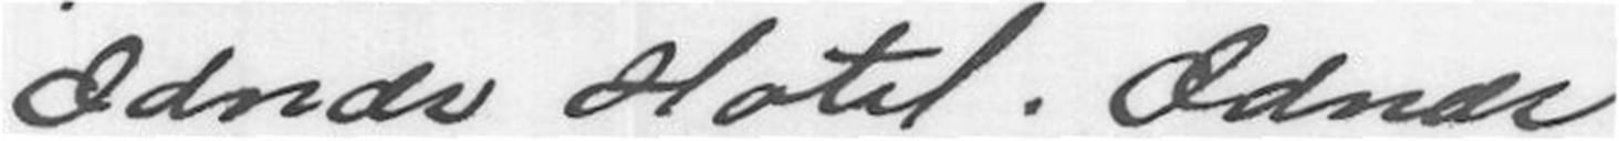Odnæs Hotel. Odnæs
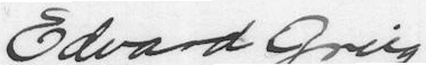Edvard Grieg
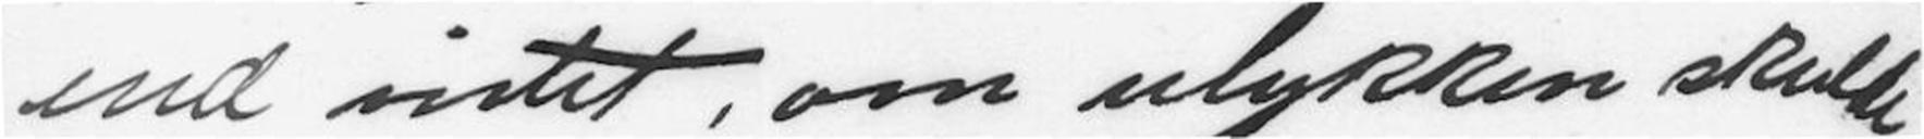end intet, om ulykken skulde
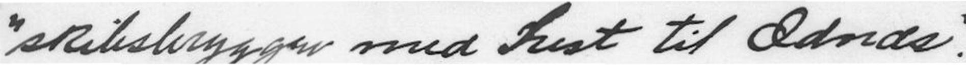"skibsbryggen med hest til Odnæs."
